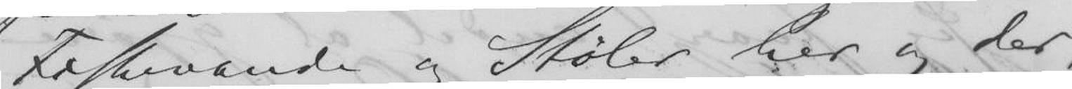Fiskevande og Støler her og der,
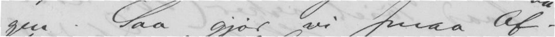gen. Saa gjør vi smaa Af-
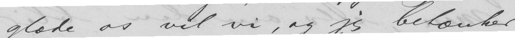glæde os vil vi, jeg betænker
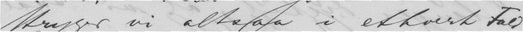stryger vi altsaa i ethvert Fald
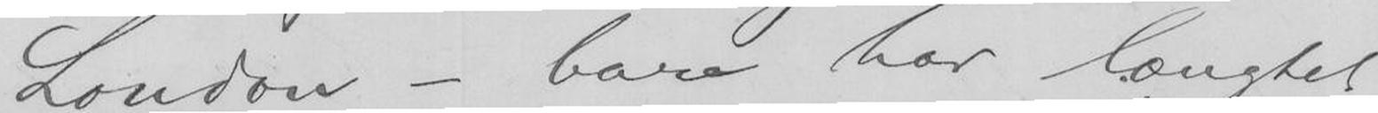London - bare har længtet
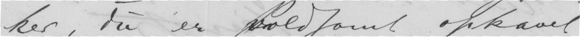ker, Du er voldsomt opkavet
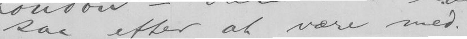saa efter at være med.
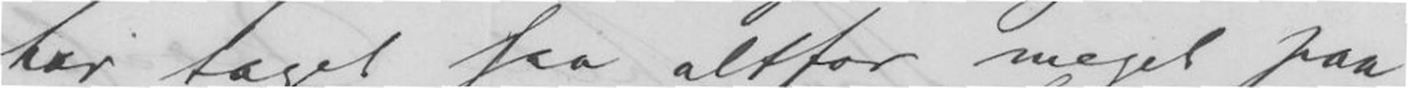har taget saa altfor meget paa
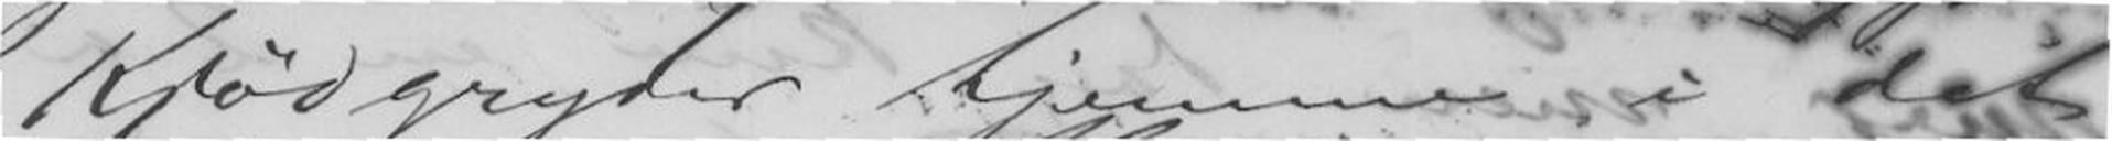Kjødgryder hjemme i det
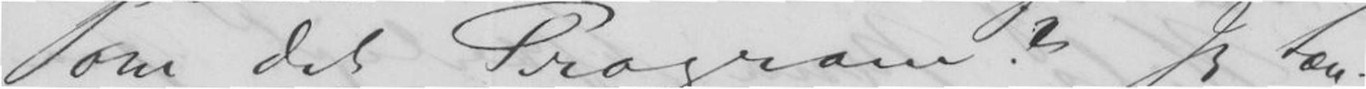om det Program? Jeg tæn-
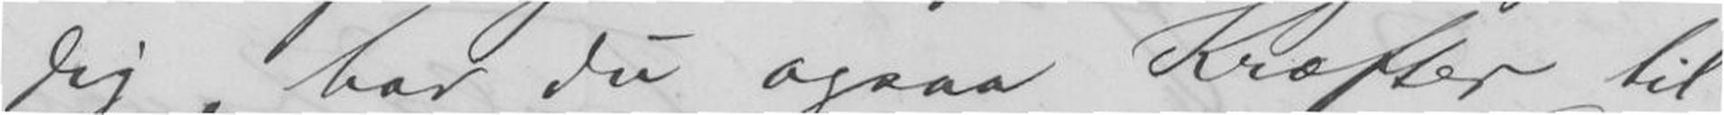Dig, har Du ogsaa Kræfter til
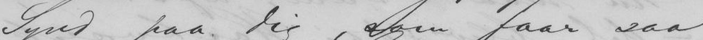Synd paa Dig, som faar saa
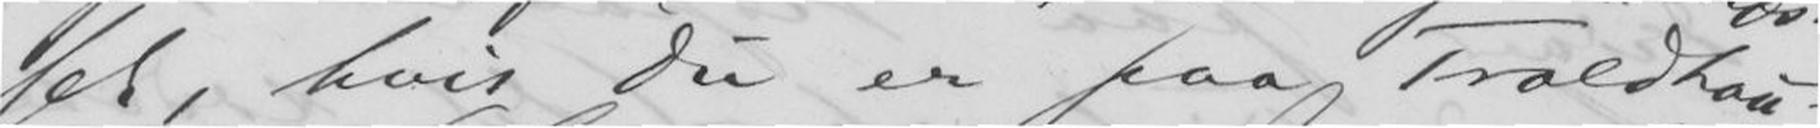set, hvis Du er paa Troldhau-
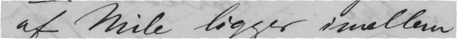af Mile ligger imellem
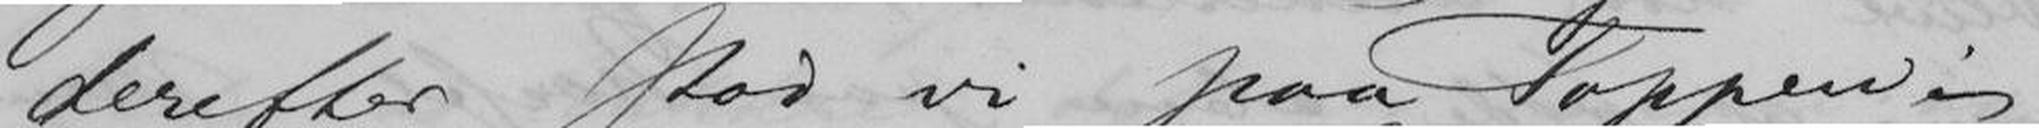derefter stod vi paa Toppen i
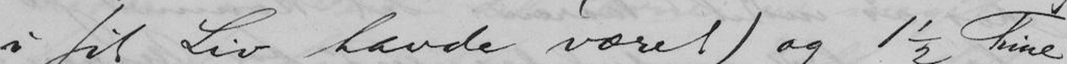i sit Liv havde været) og 1½ Time
